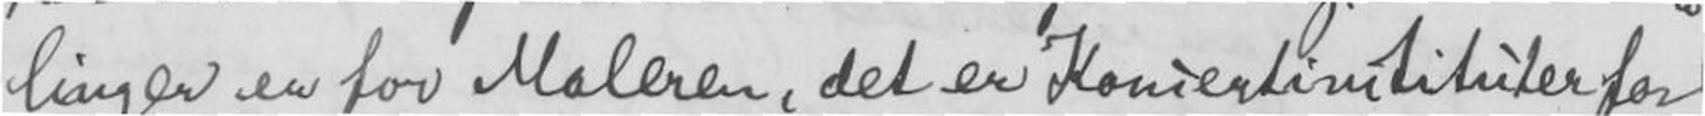linger er for Maleren, det er Koncertinstituter for
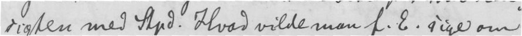sigten med Stpd. Hvad vilde man f.E. sige om
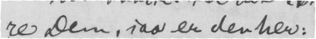re Dem, saa er den her:
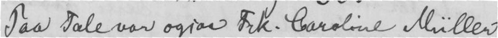Paa Tale var ogsaa Frk. Caroline Müller
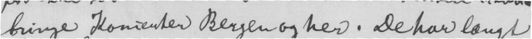bringe Koncerter Bergen og her. De har langt
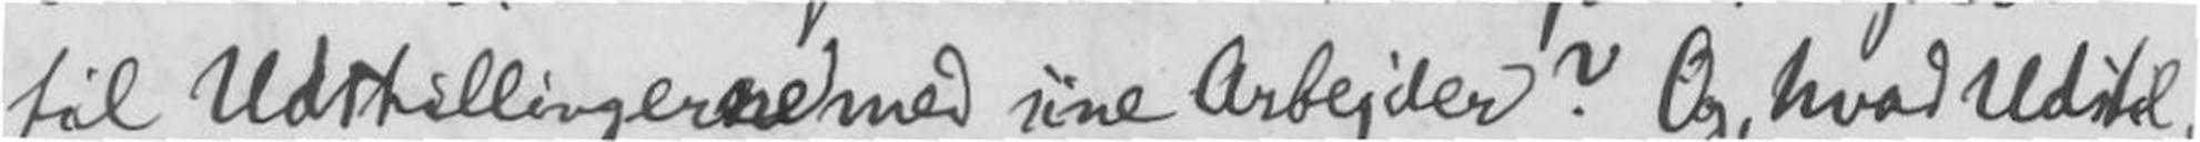til Udstillingerne med sine Arbejder? Og, hvad Udstil-
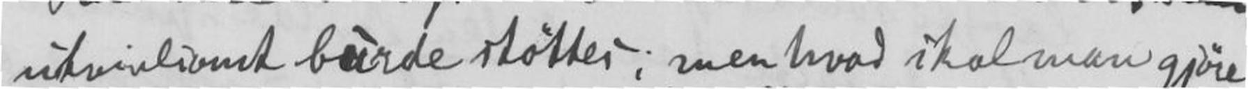utvivsomt burde støttes; men hvad skal man gjøre
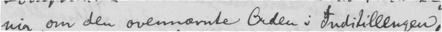nig om den ovennæmte Orden i Indstillingen,
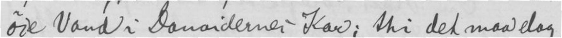øse Vand i Danoidernes Kar; thi det maa dog,
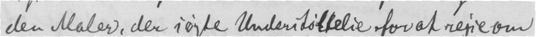den Maler, der søgte Understøttelse for at rejse om
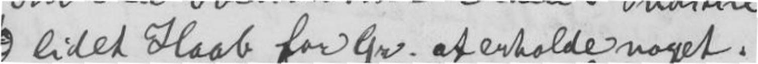lidet Haab for Gr. at erholde noget.
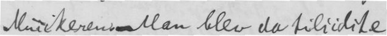Musikerens. Man blev da tilsidst e-
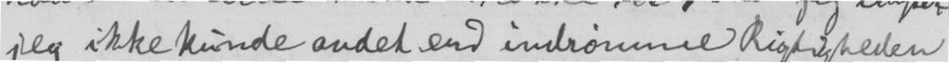jeg ikke kunde end indrømme Rigtigheden
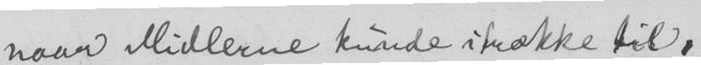naar Midlerne kunde strække til, uagtet
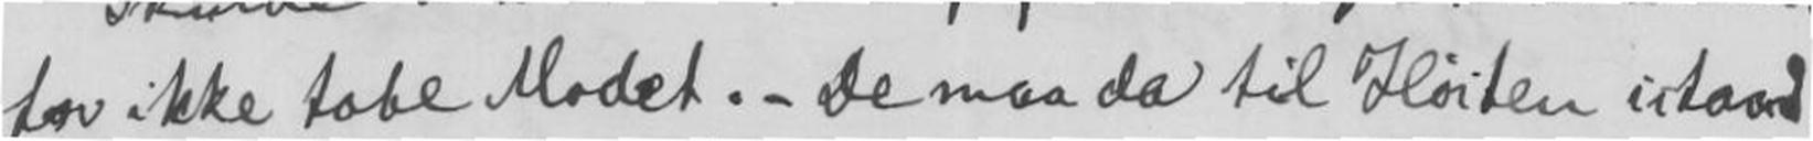for ikke tabe Modet. - De maa da til Høsten istand-
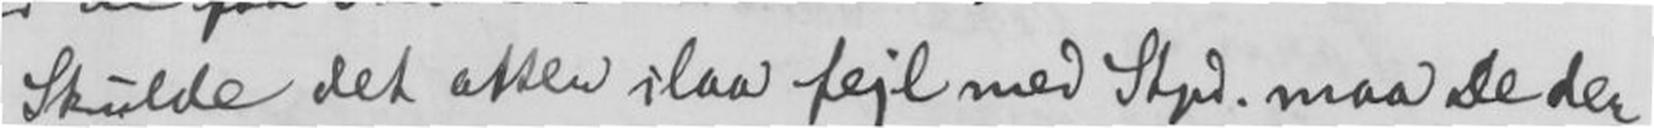Skulde det atter slaa fejl med Stpd. maa De der
